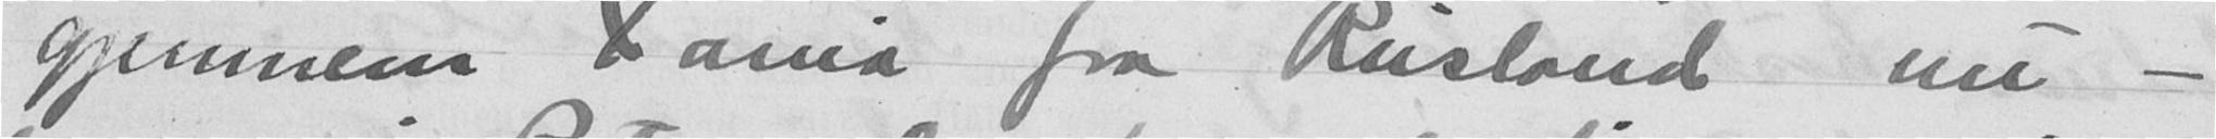gjennem Xania fra Rusland nu -
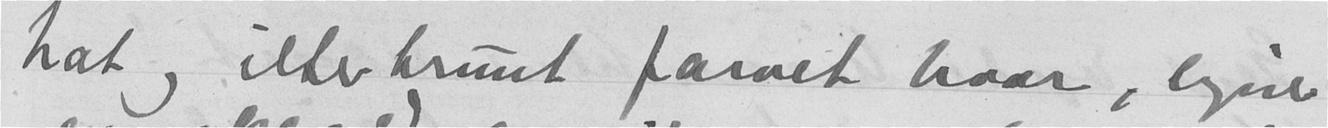hat og ilterbrunt farvet haar, ligne
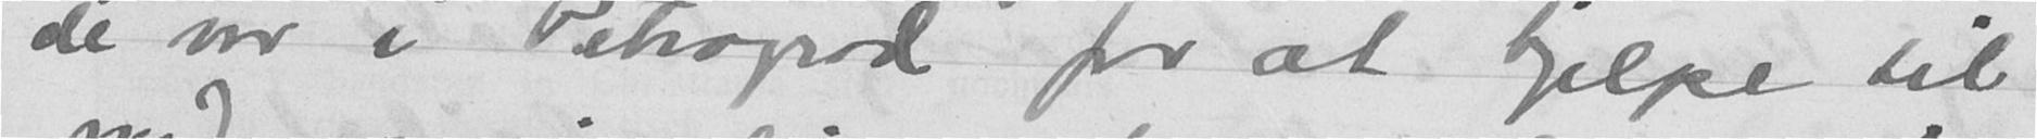de var i Petrograd for at hjelpe til
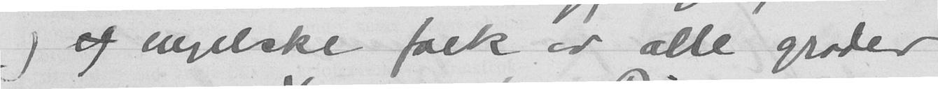og ef engelske folk av alle grader
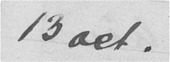13 oct.
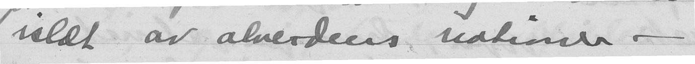islæt av alverdens nationer -
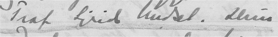Traf Sigrid Undset. Hun
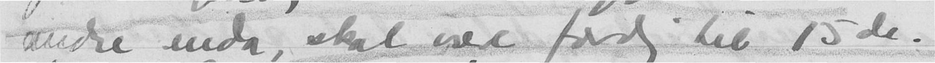andre enda, skal være færdig til 15de.
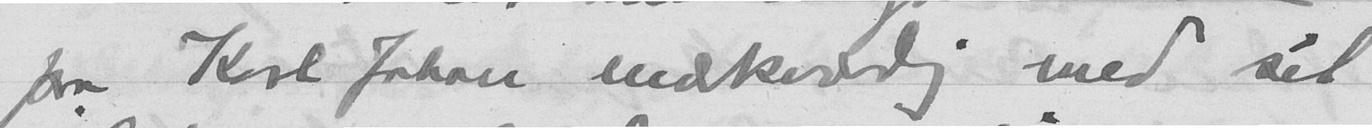paa Karl Johan mærkverdig med sit
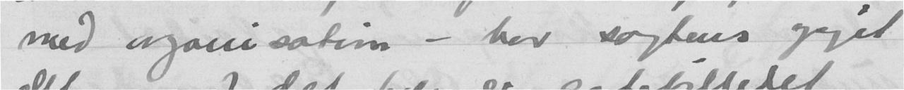med organisation - har sagtens opgit
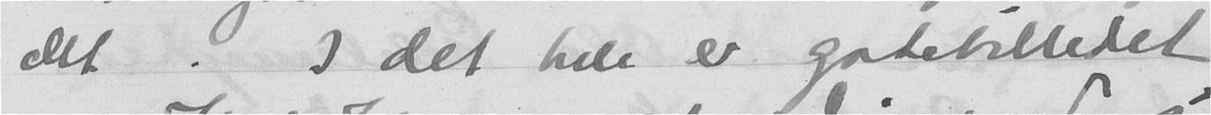det. I det hele er gatebilledet
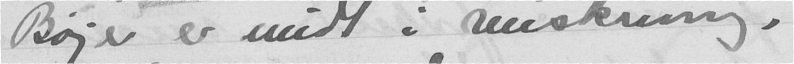Bøjer er midt i renskriving,
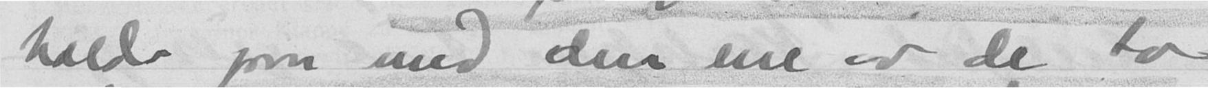holder paa med den ene av de to
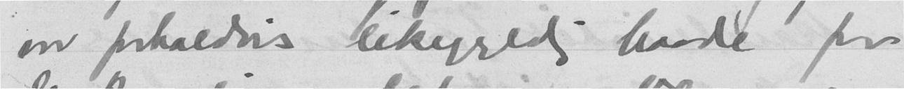var forholdsvis likegyldig baade for
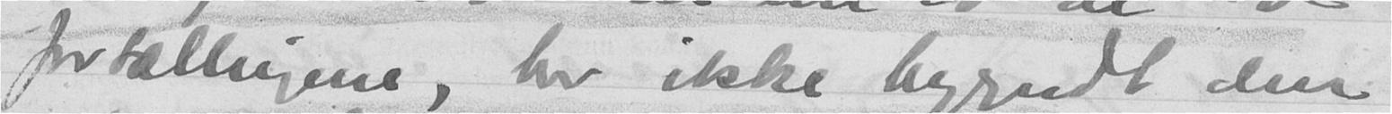fortællingene, har ikke begyndt den
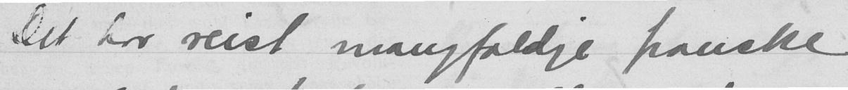Det har reist mangfoldige franske
